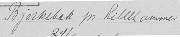Bjerkebæk pr. Lillehammer,
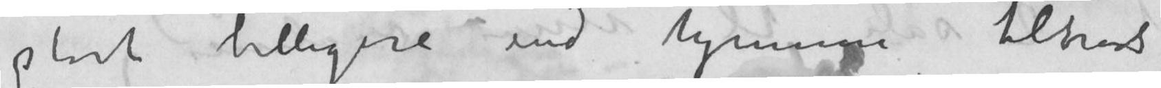stort billigere end hjemme tiltrods
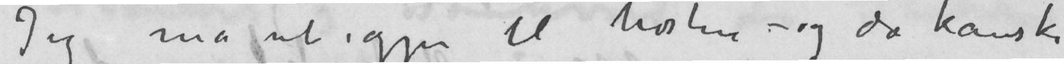Jeg må ut igjen til høsten – og da kanske
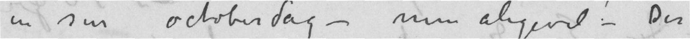en sur octoberdag – men aligevel–! Der
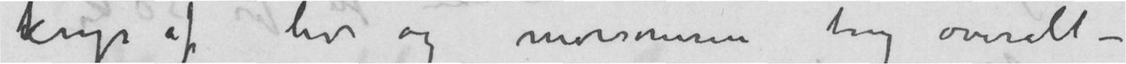kryr af liv og morsomme ting overalt –
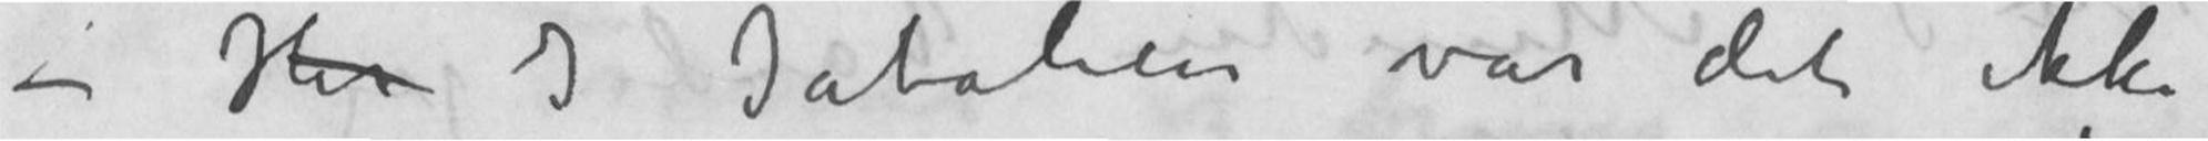– Her I Italien var det ikke
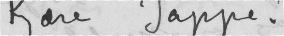Kjære Jappe!
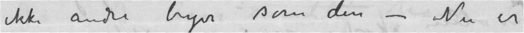ikke andre byer som den – Nu er
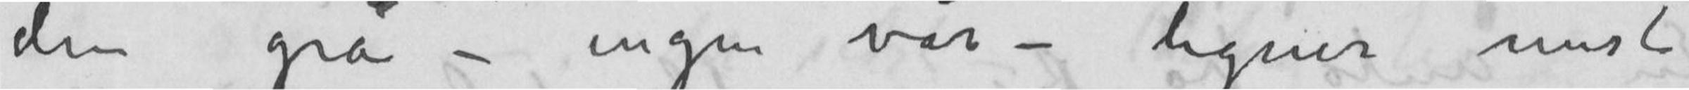den grå – ingen vår – ligner mest
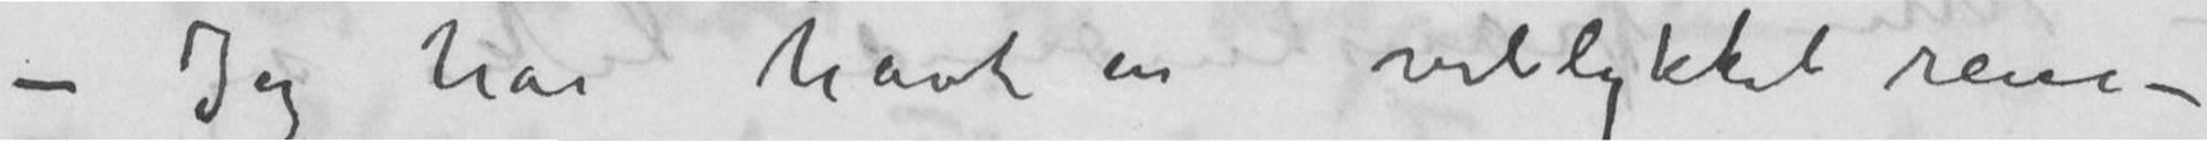– Jeg har havt en vellykket reise –
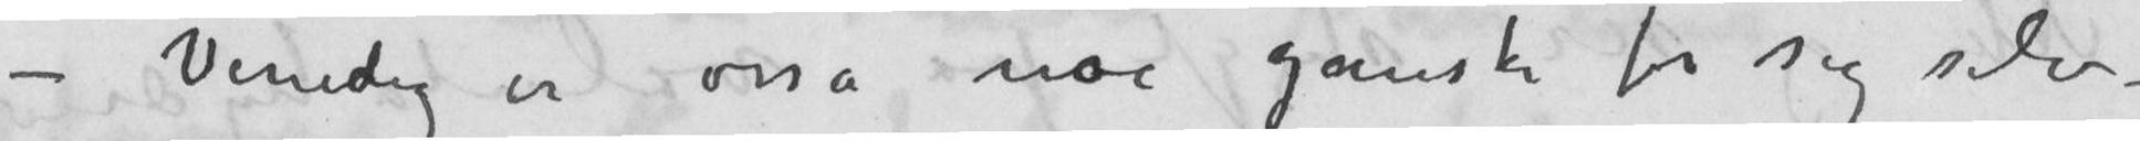– Venedig er osså noe ganske for sig selv –
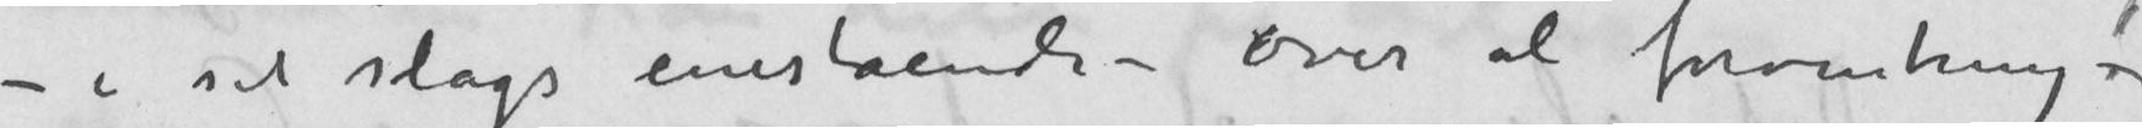– i sit slags enestående – over al forventning–!
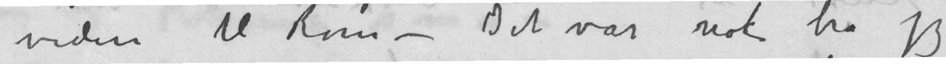videre til Rom – Det var nok bra jeg
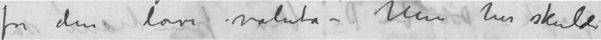for den lave valuta – Men her skulde
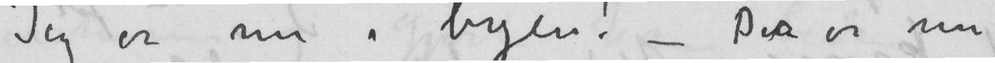Jeg er nu i byen! – Denr er nu
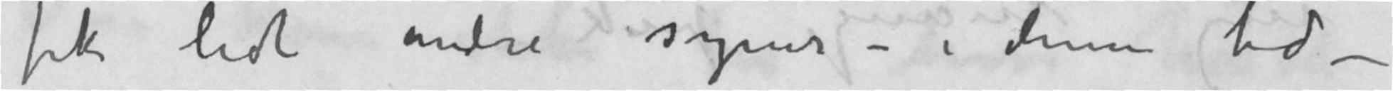fik lidt andre syner – i denne tid –
